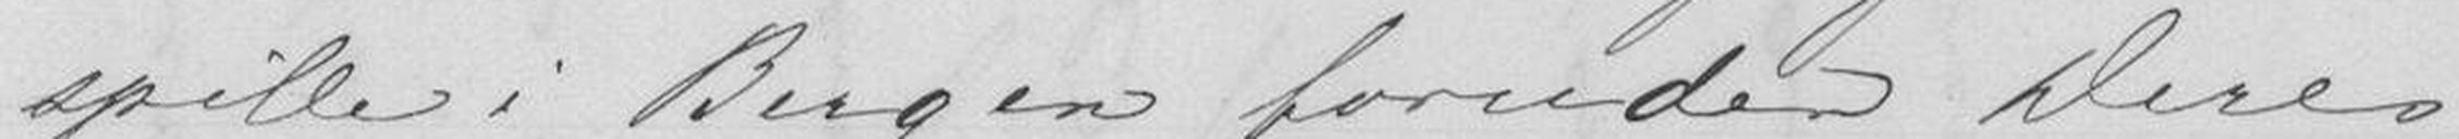spille i Bergen foruden Deres
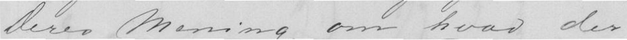Deres Mening om hvad der
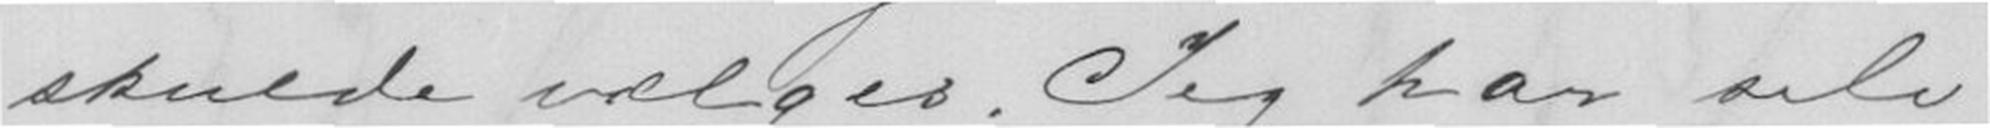skulde vælges. Jeg har selv
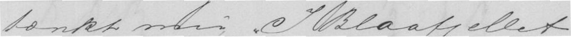tænkt mig I Blaafjellet
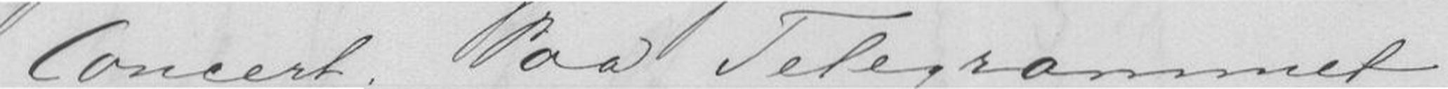Concert. Paa Telegrammet
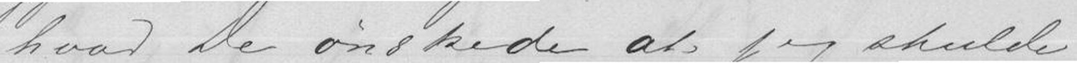hvad De ønskede at jeg skulde
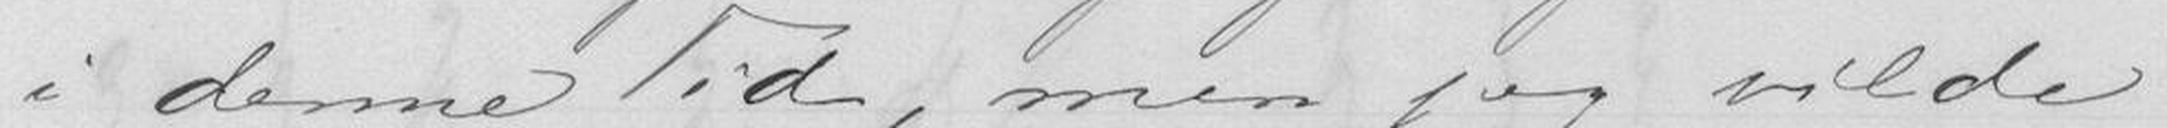i denne Tid, men jeg vilde
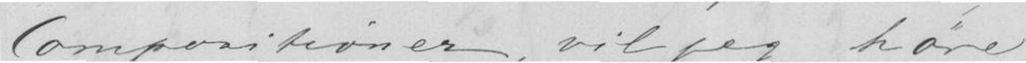Compositioner, vil jeg høre
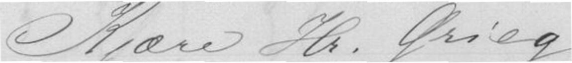Kjære Hr. Grieg
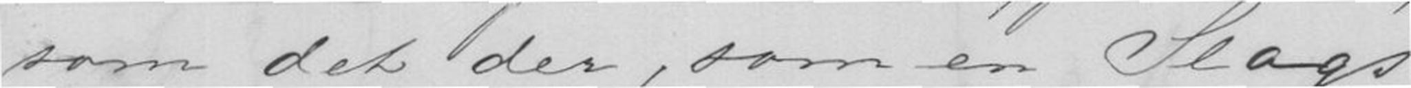som det der, som en Slags
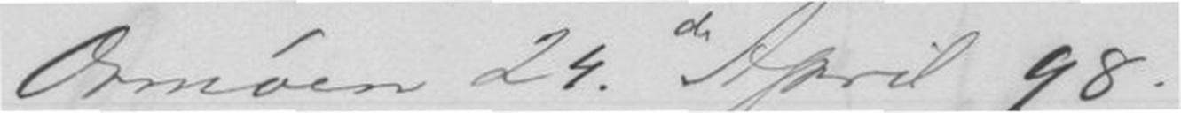Ormøen 24. deApril 98.
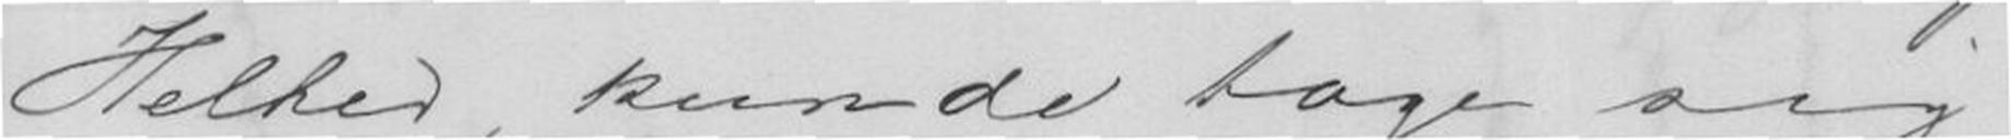Helhed, kunde tage sig
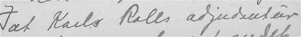af Karls Rolls adjudantur
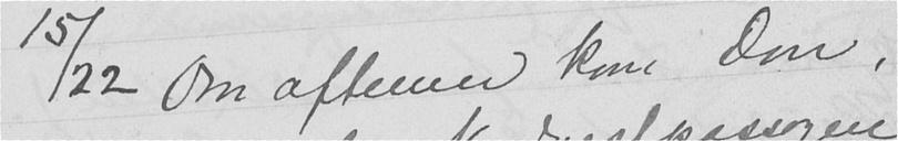15/22 Om aftenen kom Don,
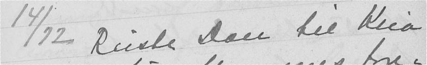14/12 Reiste Don til Kria
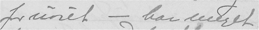fornøiet - har meget
